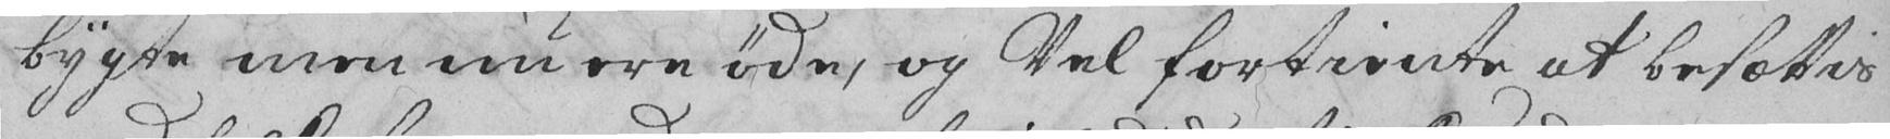bygte men nu ere øde, og Vel fortiente at besættis
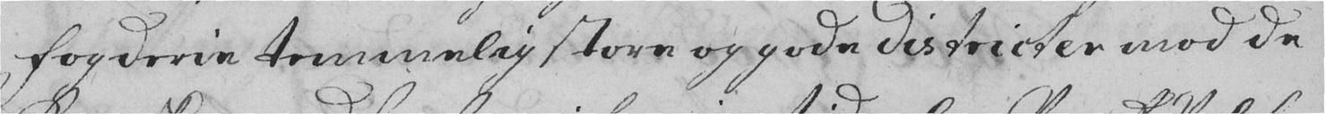Fogderie temmelig store og gode districter mod de
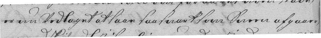er nu Vedtaget at saae saa snart som Sneen afgaaer,
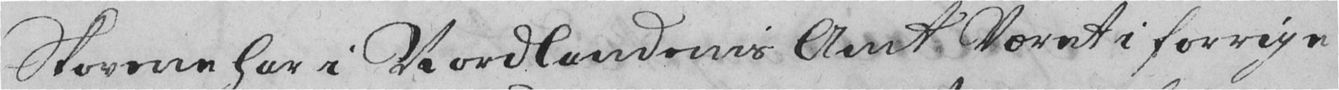Skovene har i Nordlandenis Amt Været i forrige
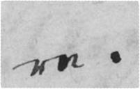re.
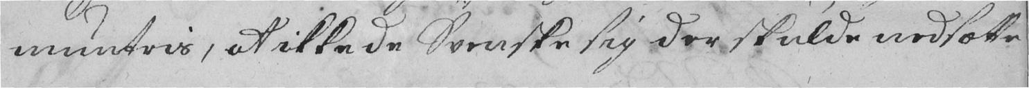muntris, at ikke de Svenske sig der skulde nedsætte.
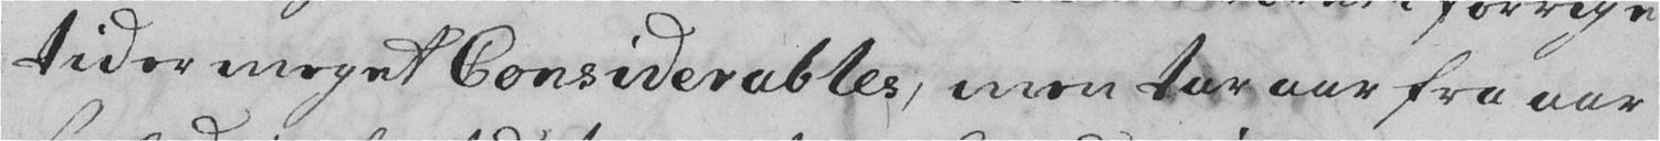tider meget Considerables, men tar aar fra aar
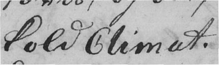kold Climat.
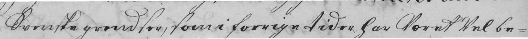Svenske grendser, som i forrige tider har Været Vel be-
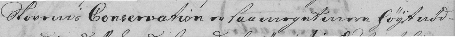Skovenis Conservation er saa meget mere høyt nød-
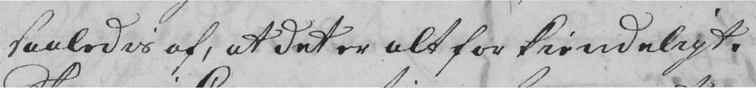saaledis af, at det er alt for kiendeligt.
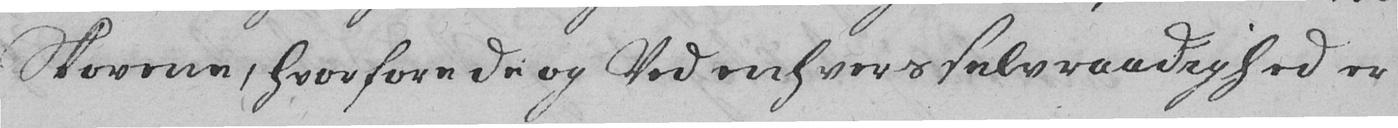Skovene, hvorfore de og Ved en hvers selvraadighed er
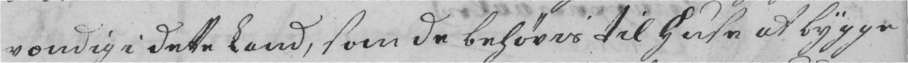vændig i dette Land, som de behøvis til Huse at bygge
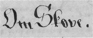Om Skove
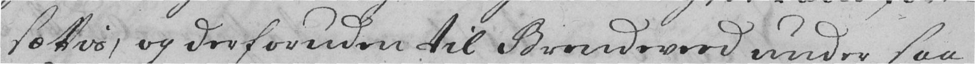sættis, og derforuden til Brendeveed under saa
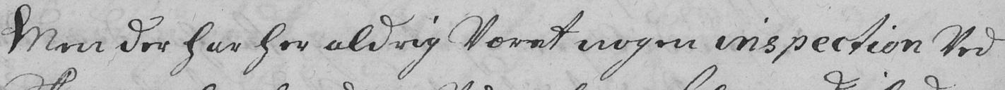Men der har her aldrig Været nogen inspection Ved
